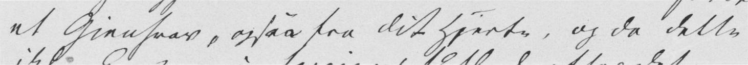et Gjensvar, ogsaa fra Dit Hjerte, og da dette
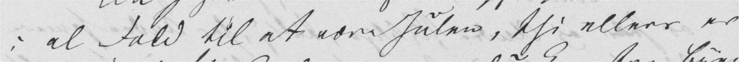i al Fald til at være Julen, thi ellers er
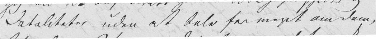Fataliteter uden at tale saa meget om dem,
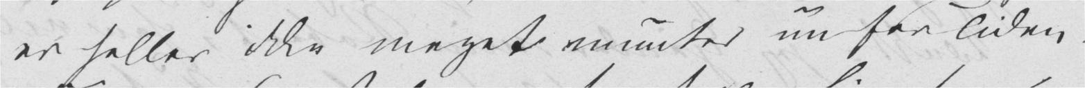er heller ikke meget munter nu for Tiden.
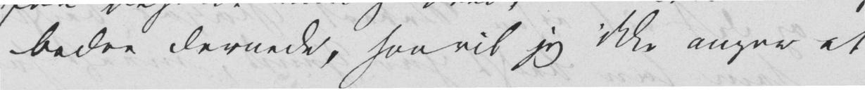bedre dernede, saa vil jeg ikke angre at
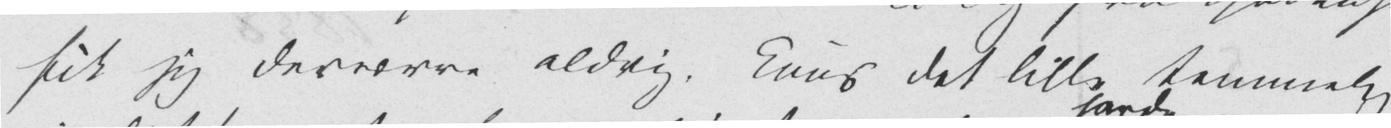fik jeg desværre aldrig. Kuns det lille temmelig
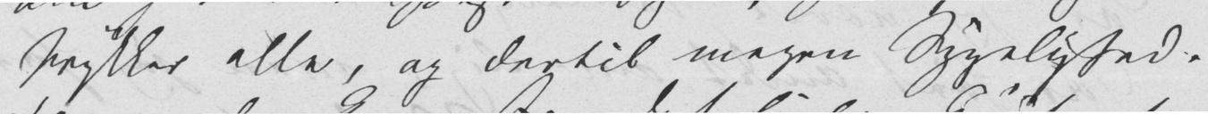trykker alle, og dertil megen Sygelighed.
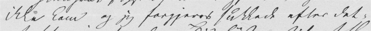ikke kom og jeg forgjeves sukkede efter det,
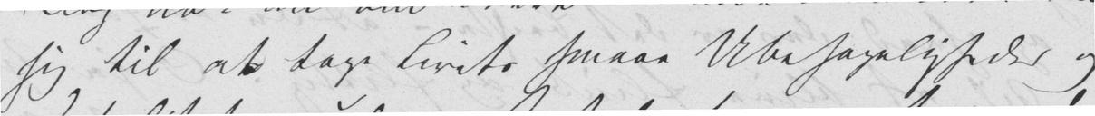sig til at tage Livets smaae Ubehageligheder og
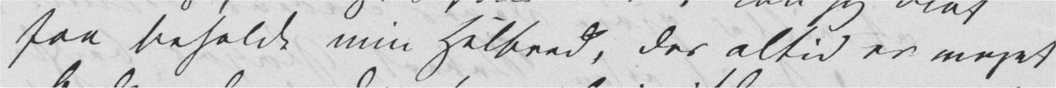faa beholde min Helbred, der altid er meget
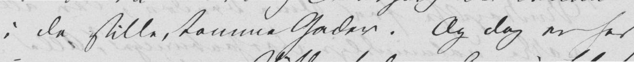i de stille, tomme Gader. Og dog er her
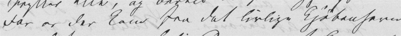For os der kom fra det livlige Kjøbenhavn
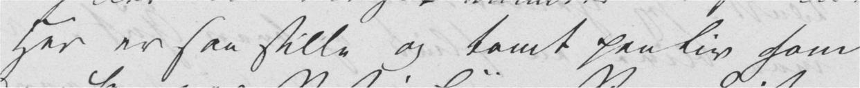Her er saa stille og tomt paa Liv som
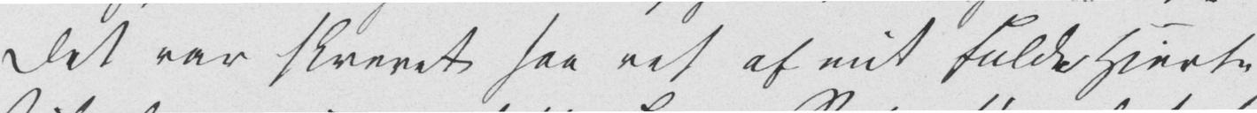Det var skrevet saa ret af mit fulde Hjerte
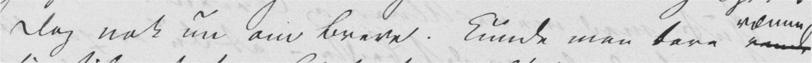Dog nok nu om Breve. Kunde man bare vende
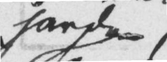havde
